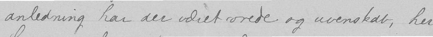anledning har der været vrede og uvenskab, her
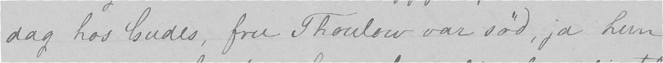dag hos Gudes, fru Thoulow var sød, ja hun
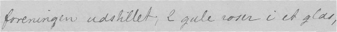foreningen udstillet, 2 gule roser i et glas,
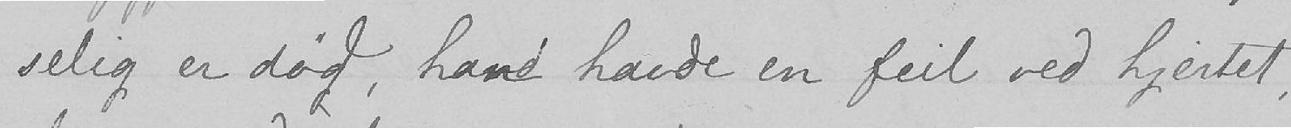selig er død, han havde en feil ved hjertet,
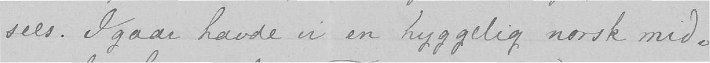sees. Igaar havde vi en hyggelig norsk mid-
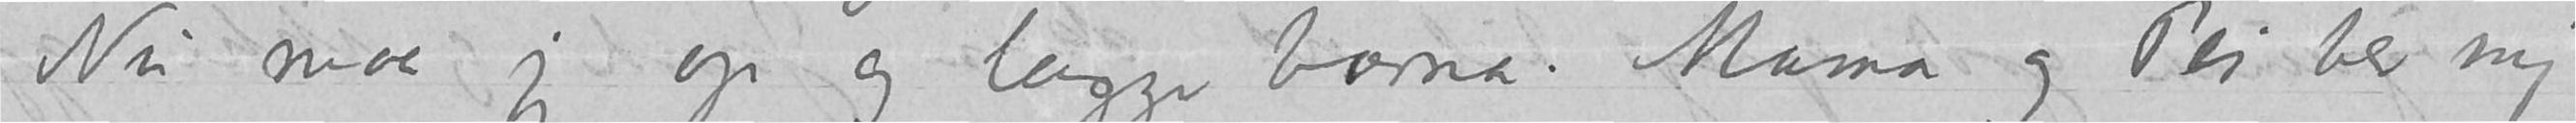Nu maa jeg op og lægge barna. Mama og Pei ber mig
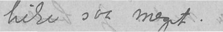hilse saa meget.
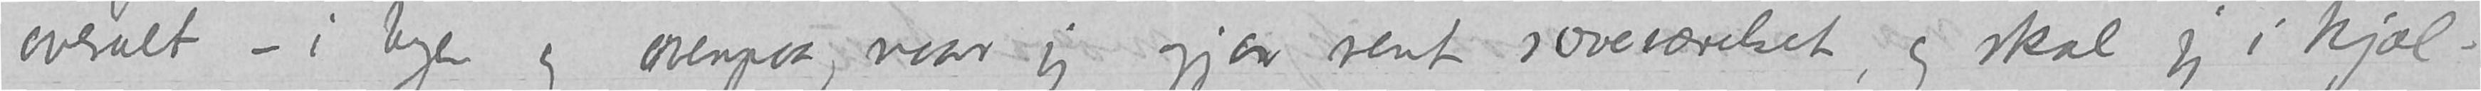overalt – i byen og ovenpaa, naar jeg gjør rent soveværelset, og skal jeg i kjæl-
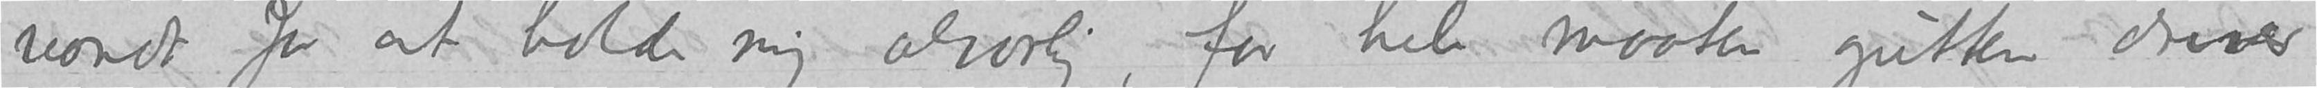vondt for at holde mig alvorlig, for hele maaten gutten driver
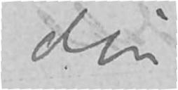din
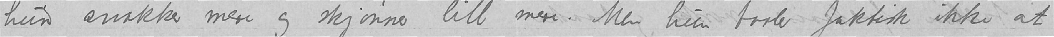hun snakker mere og skjønner litt mere. Men hun taaler faktisk ikke at
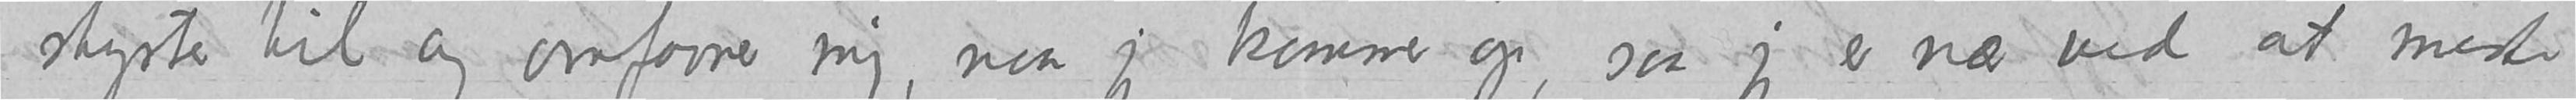styrter til og omfavner mig, naar jeg kommer op, saa jeg er nær ved at miste
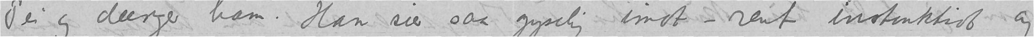Pei og dænger ham. Han sier saa gyselig imot – rent instinktivt og
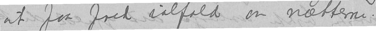at faa fred ialfald om nætterne.
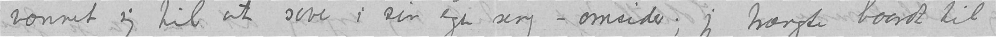vænnet sig til at sove i sin egen seng – omsider; jeg trængte haardt til
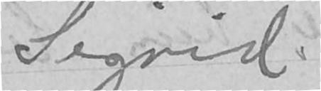Sigrid
